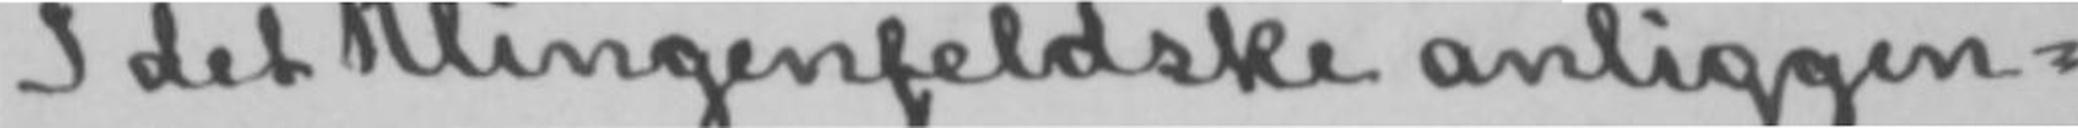I det Klingenfeldske anliggen-
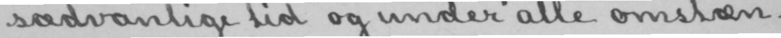sædvanlige tid og under alle omstæn-
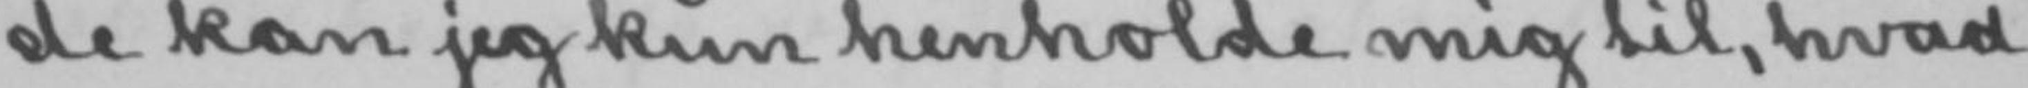de kan jeg kun henholde mig til, hvad
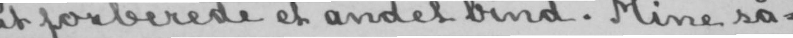at forberede et andet bind. Mine så-
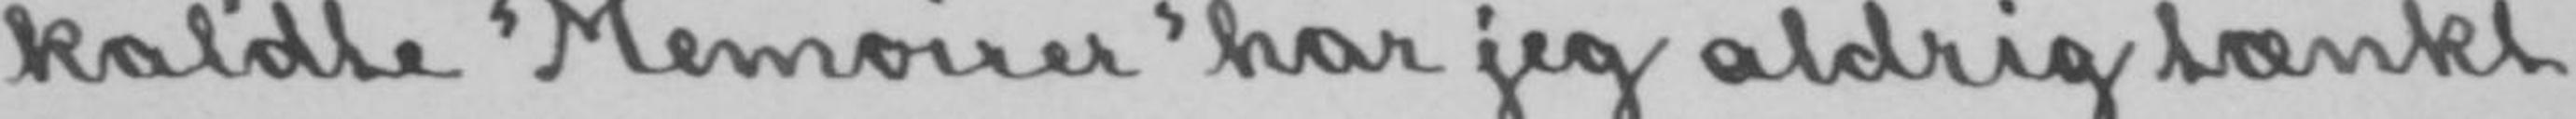kaldte «Memoirer» har jeg aldrig tænkt
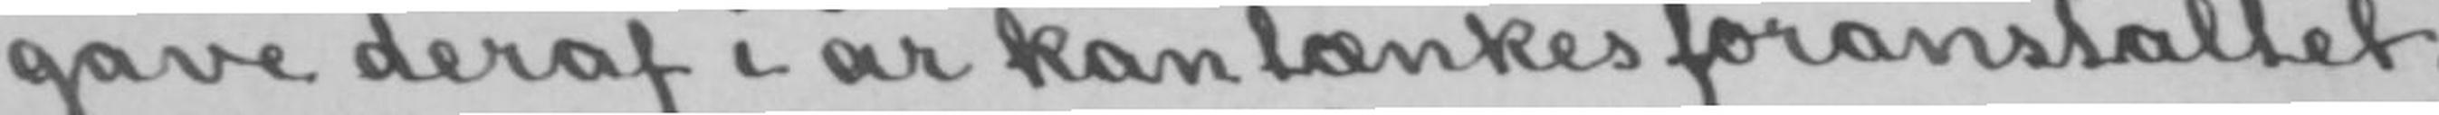gave deraf i år kan tænkes foranstaltet.
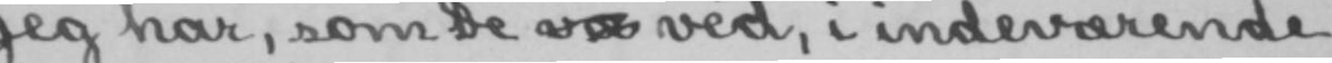Jeg har, som De væ véd, i indeværende
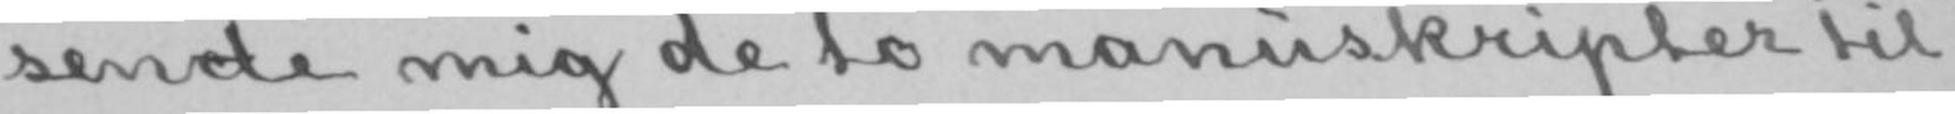sende mig de to manuskripter til
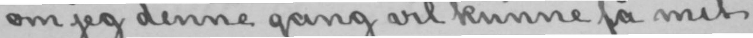om jeg denne gang vil kunne få mit
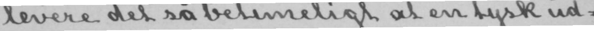levere det så betimeligt at en tysk ud-
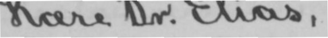Kære Dr. Elias,
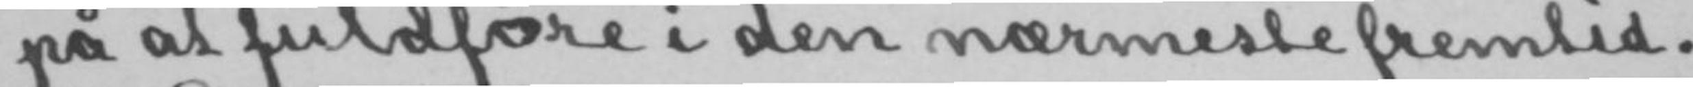på at fuldføre i den nærmeste fremtid.
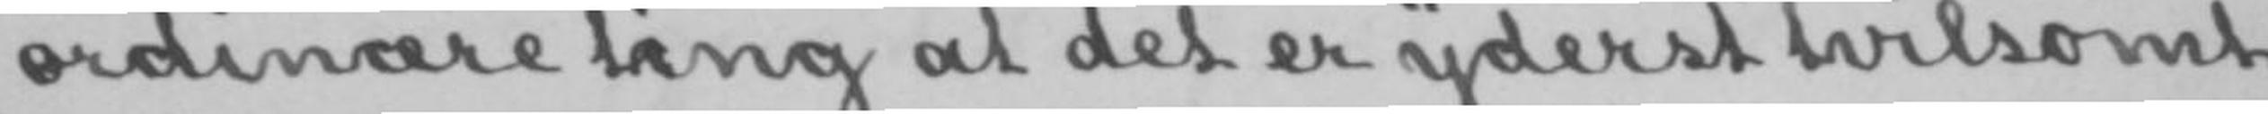ordinære ting at det er yderst tvilsomt
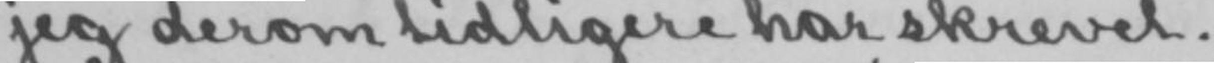jeg derom tidligere har skrevet.-
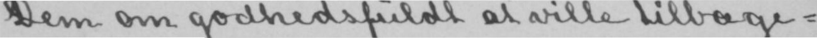Dem om godhedsfuldt at ville tilbage-
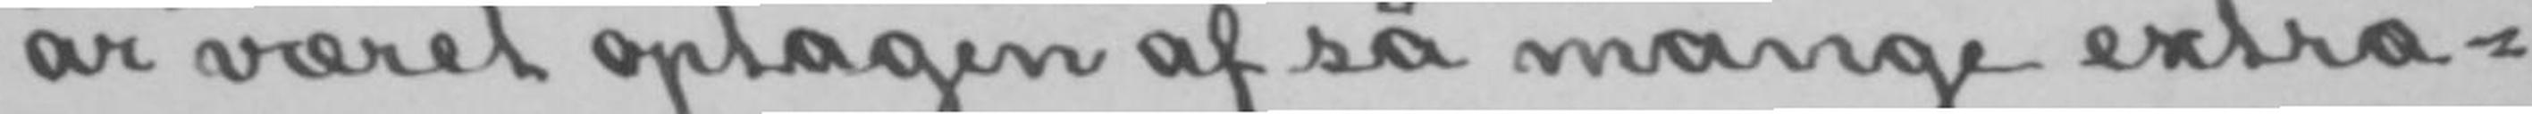år været optagen af så mange extra-
